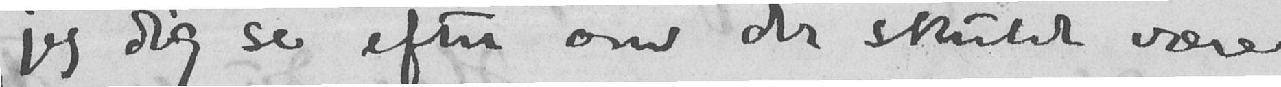jeg dig se efter om der skulde være
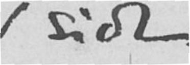side
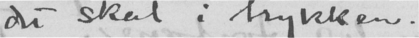det skal i trykken.
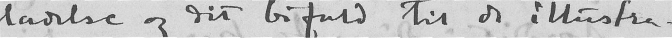ladelse og dit bifald til de illustra-
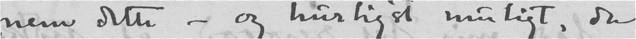nem dette - og hurtigst muligt, da
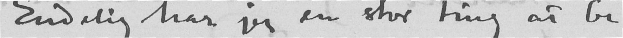Endelig har jeg en stor ting at be
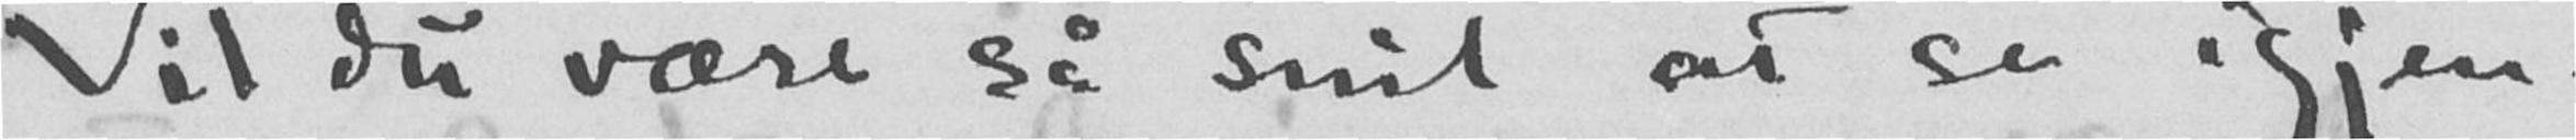Vil du være så snil at se igjen-
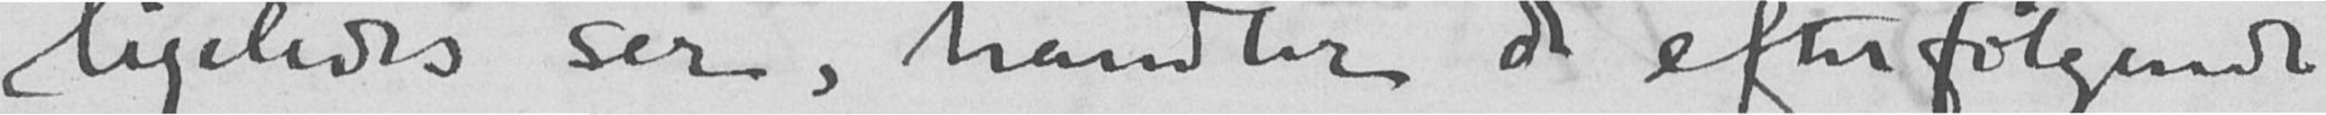ligeledes ser, handler de efterfølgende
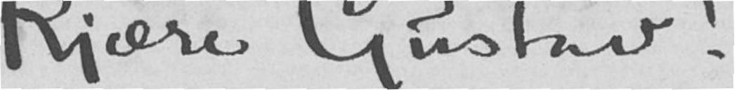Kjære Gustav!
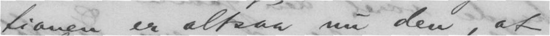tionen er atsaa nu den, at
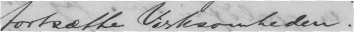fortsætte Virksomheden.
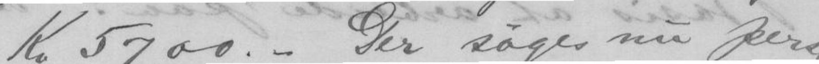Kr 5700. - Der søges nu person-
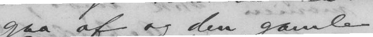gaa af og den gamle
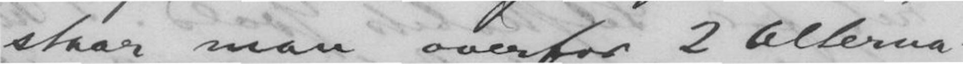staar man overfor 2 Alterna-
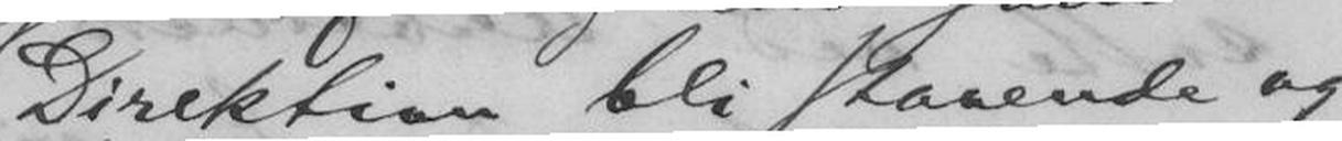Direktion bli staaende og
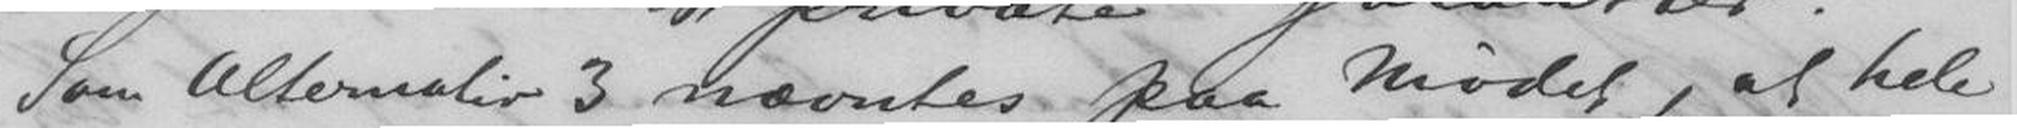Som Alternativ 3 nævntes paa Mødet, at hele
